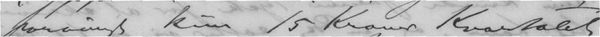forøvrigt kun 15 Kroner Kvartalet.
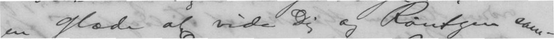en glæde at vide Dig og Röntgen sam-
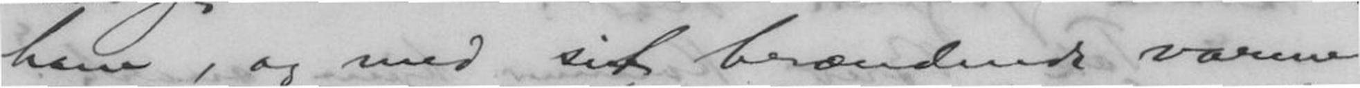ham, og med sit brændende varme
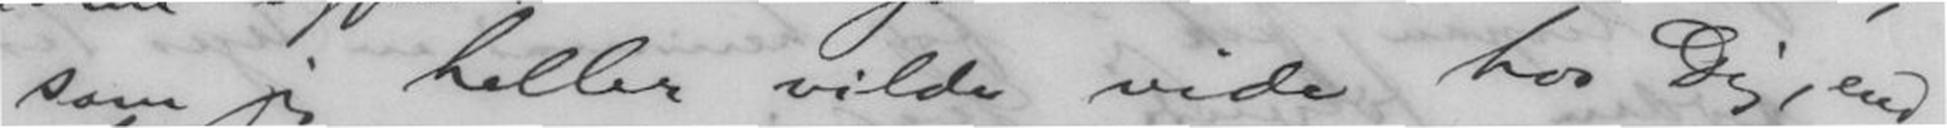som jeg heller vilde vide hos Dig, end
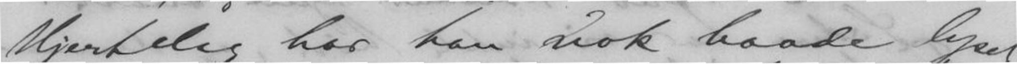Hjertelag har han nok baade lyset
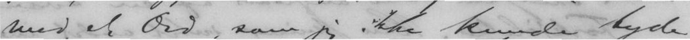med et Ord, som jeg ikke kunde tyde,
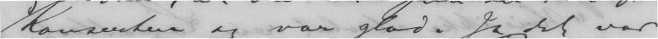Konserten og var glad. Ja det var
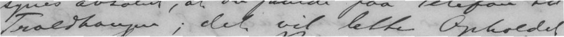Troldhaugen; det vil lette Opholdet
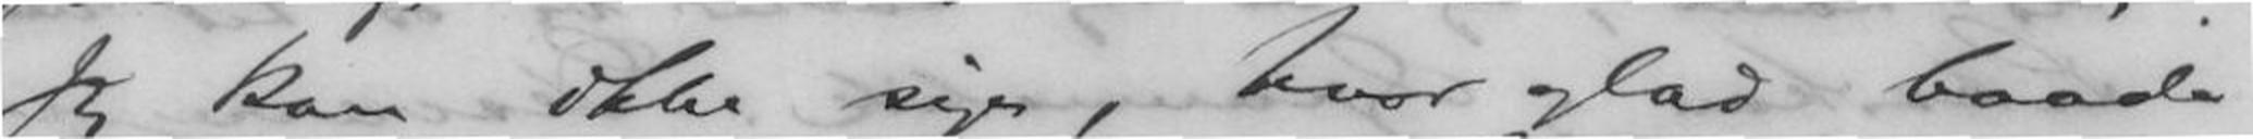Jeg kan ikke sige, hvor glad baade
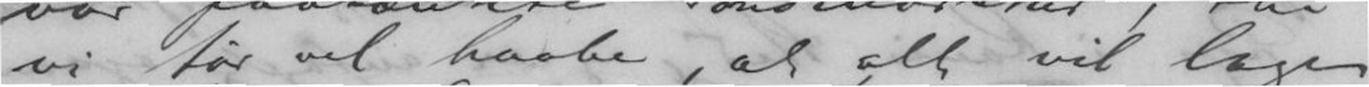vi tør vel haabe, at alt vil lage
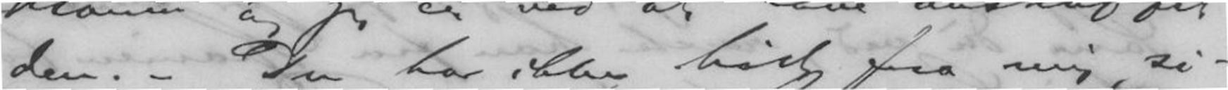den. - Du har ikke hørt fra mig si-
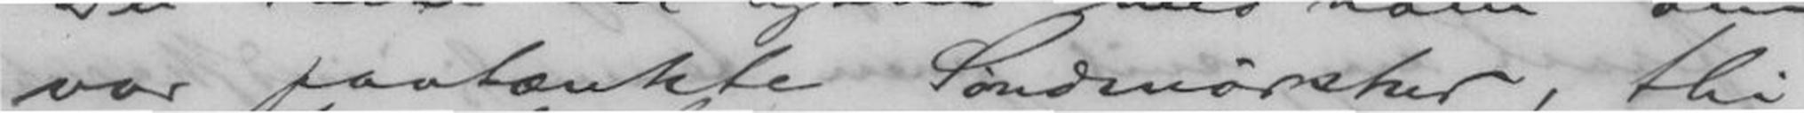vor paatænkte Søndmørstur, thi
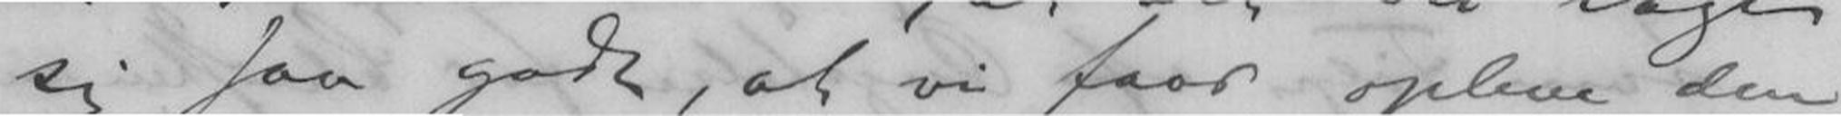sig saa godt, at vi faar opleve den
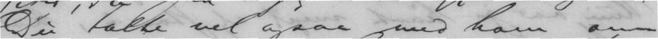Du talte vel ogsaa med ham om
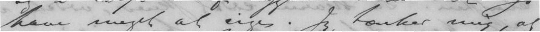have meget at sige. Jeg tænker mig, at
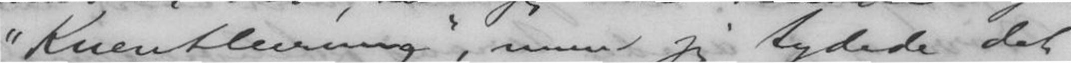"Kuentlevning", men jeg tydede det
